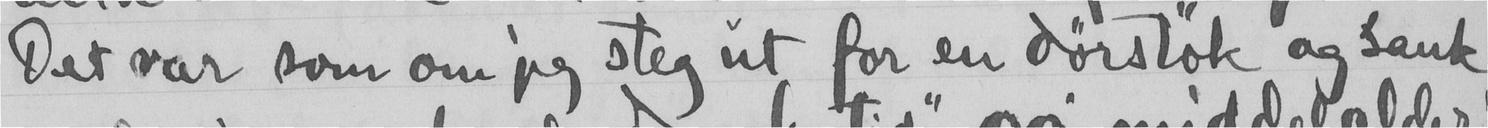Det var som om jeg steg ut for en dørstok og sank
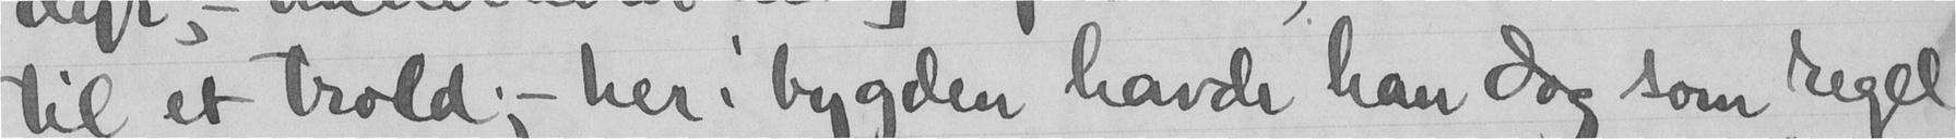til et trold; - her i bygden havde han dog som regel
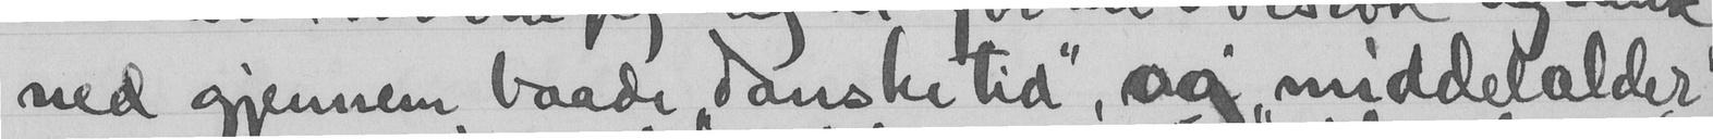ned gjennem baade "danske tid", og "middelalder"
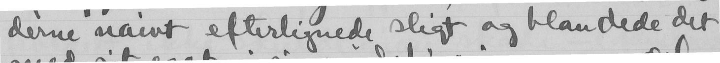derne naivt efterlignede sligt og blandede det
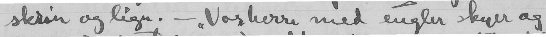skrin og lign. - "Vorherre med engler skyer og
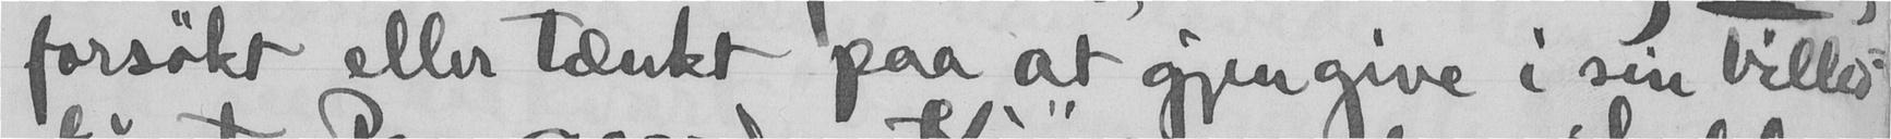forsøkt eller tænkt paa at gjengive i sin billed-
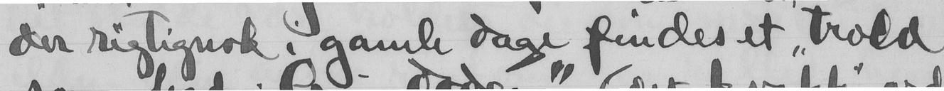der sigtignok i gamle dage findes et "trold"
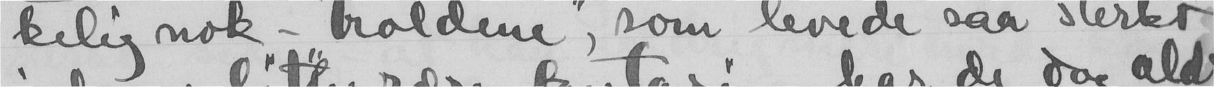kelig nok - troldene", som levede saa sterkt
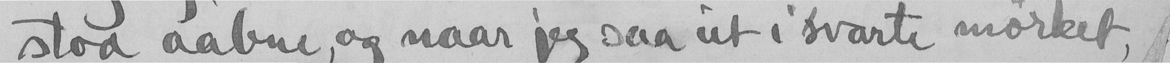stod aabne, og naar jeg saa ut i svarte mørket,
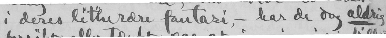i deres litherære fantasi, - har de dog aldrig
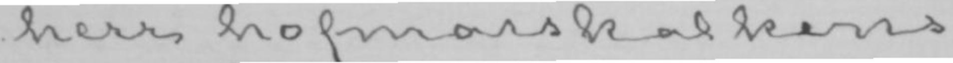herr hofmarskalkens
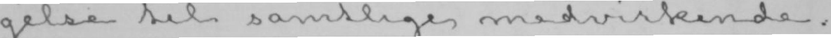gelse til samtlige medvirkende.
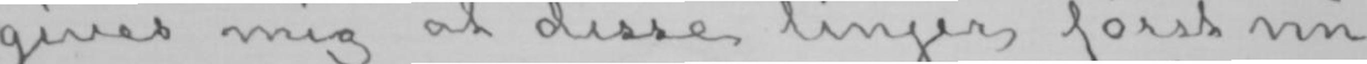gives mig at disse linjer først nu
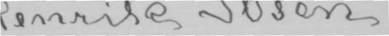Henrik Ibsen.
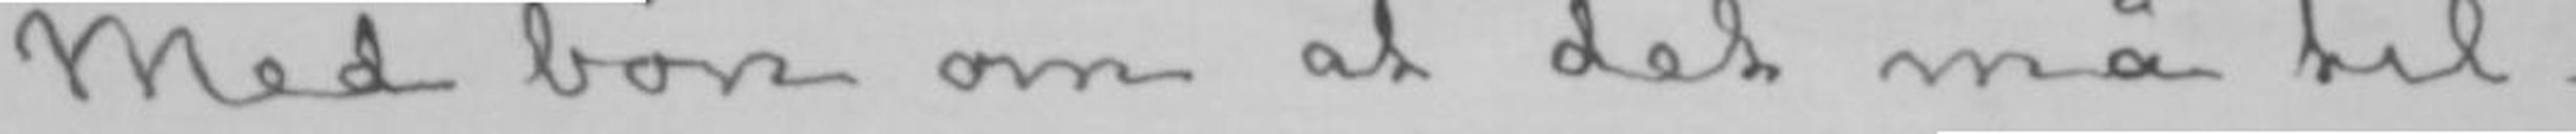Med bøn om at det må til-
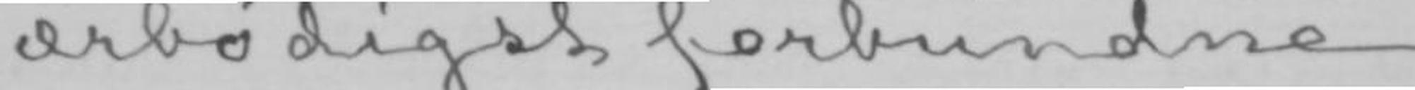ærbødigst forbundne
